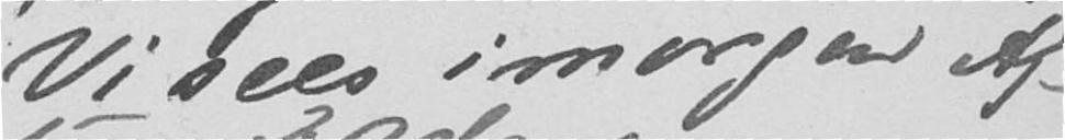Vi sees i morgen Af-
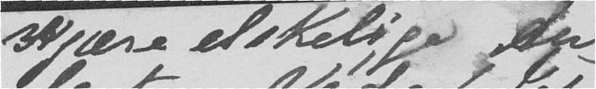Kjære elskelige Au-
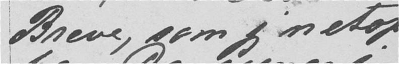Breve, som jeg netop
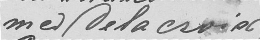med Delacroix'
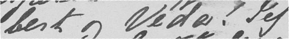bert og Veda! Jeg
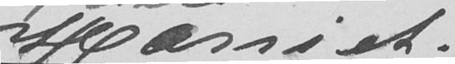Harriet.
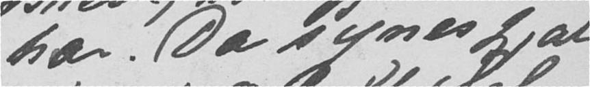har. Da synes jeg, at
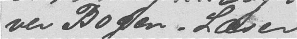ver Bogen. Læser
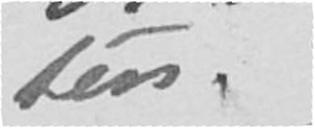ten.
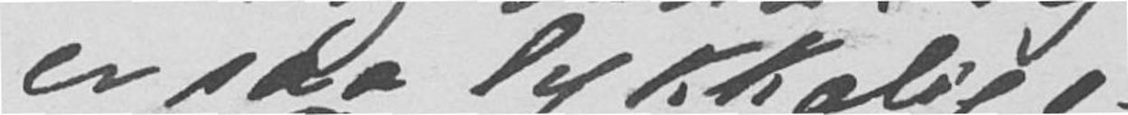er saa lykkelig o-
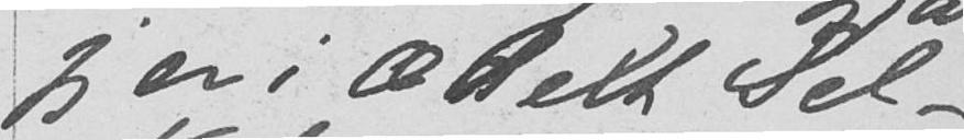jeg er i ædelt sel-
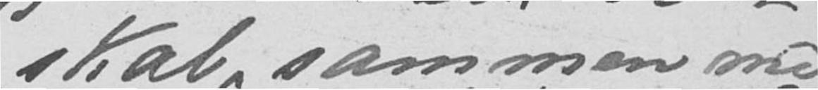skab, sammen med
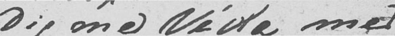Dig med Véda med
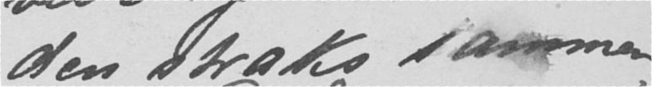den straks sammen
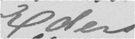Eders
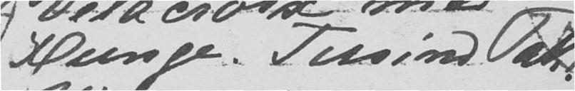Runge. Tusind Tak!
